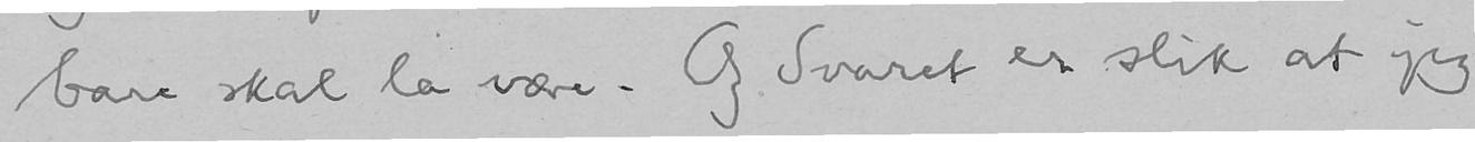bare skal la være. Og Svaret er slik at jeg
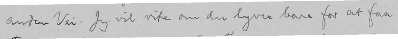anden Vei. Jeg vil vite om du lyver bare for at faa
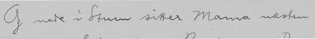Og nede i Stuen sitter Mama næsten
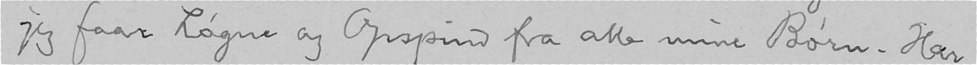jeg faar Løgne og Opspind fra alle mine Børn. Har
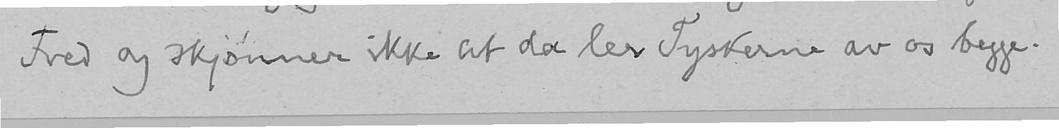Fred og skjønner ikke at da ler Tyskerne av os begge.
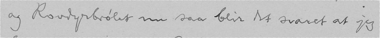og Rovdyrbrølet nu saa blir det svaret at jeg
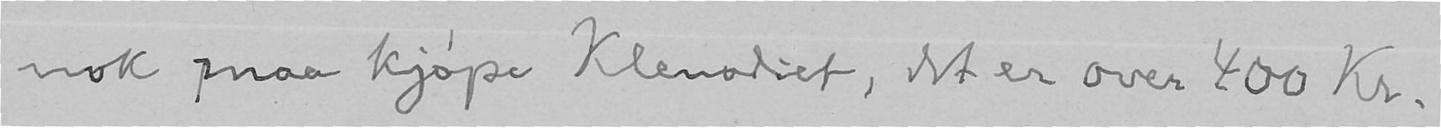nok maa kjøpe Klenodiet, det er over 400 Kr.
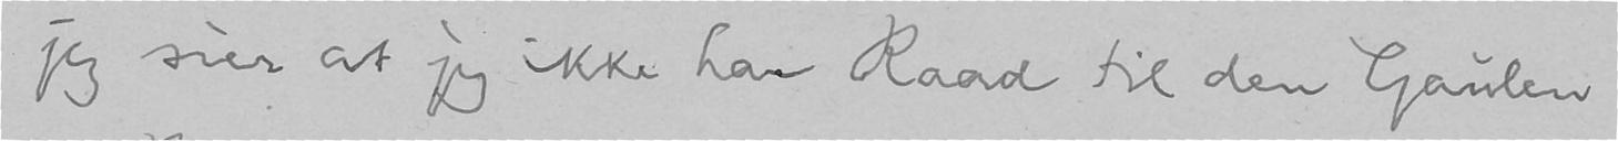jeg sier at jeg ikke har Raad til den Gaulen
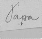Papa
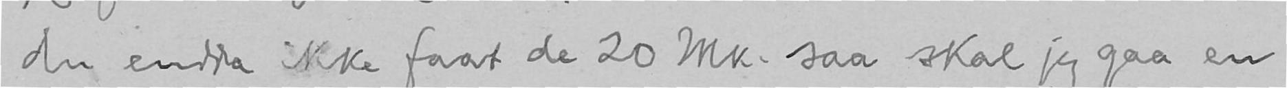du endda ikke faat de 20 Mk. saa skal jeg gaa en
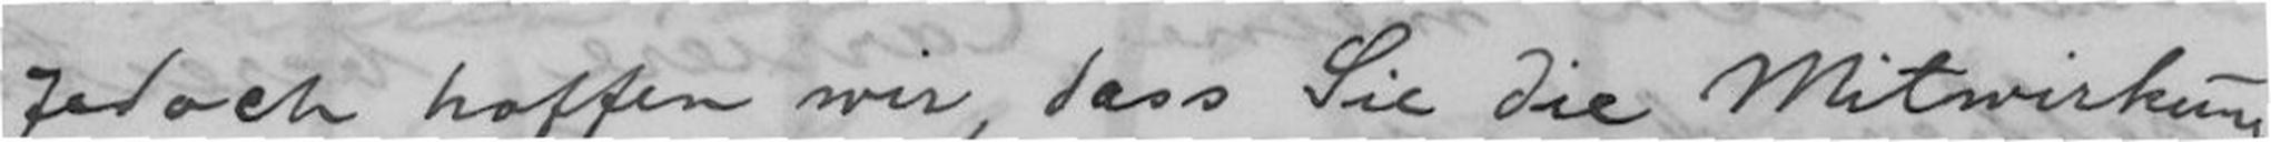Jedoch hoffen wir, dass Sie die Mitwirkung
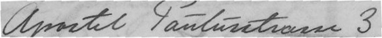Apostel Paulusstrasse 3
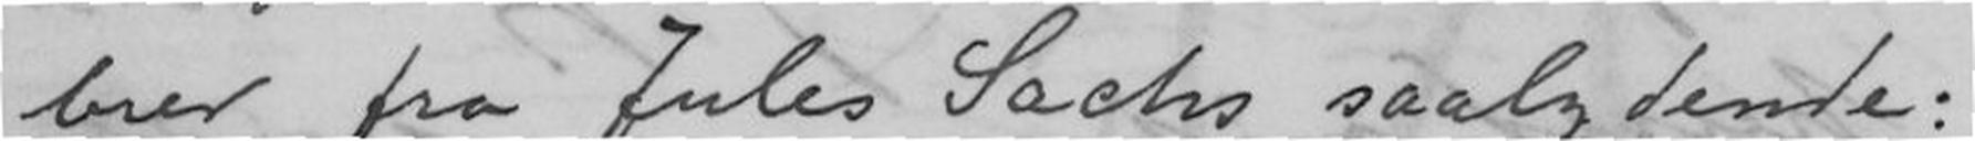brev fra Jules Sachs saalydende:
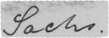Sachs.
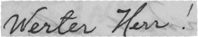Werter Herr!
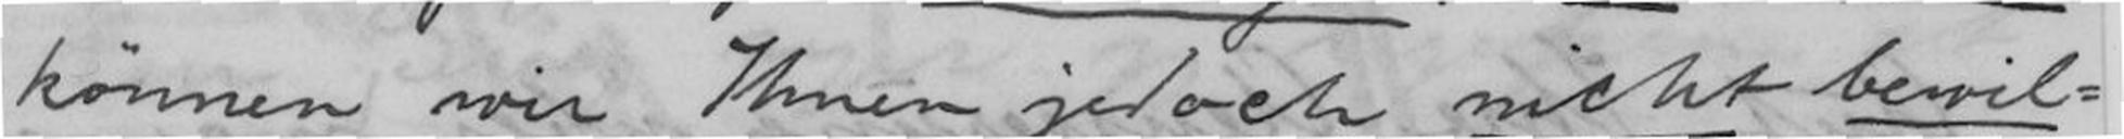können wir Ihnen jedoch nicht bewil-
